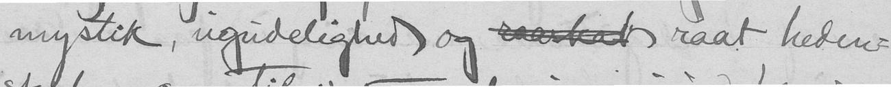mystik ugudelighed og  raat heden-
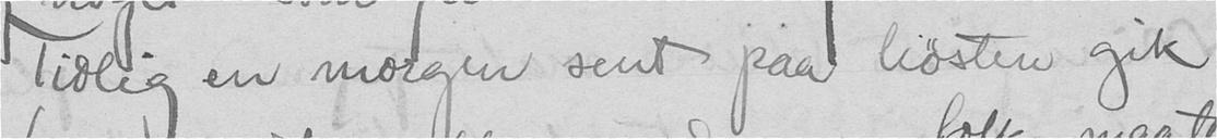Tidlig en morgen sent paa høsten gik
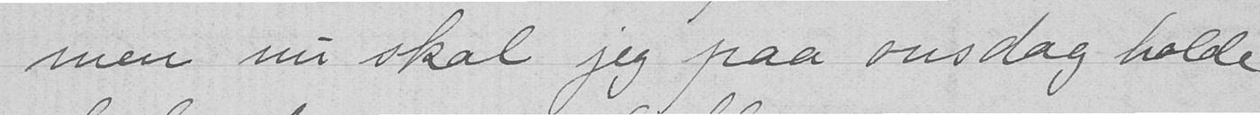men nu skal jeg paa onsdag holde
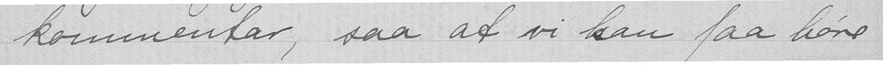kommentar, saa at vi kan faa høre
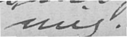mig.
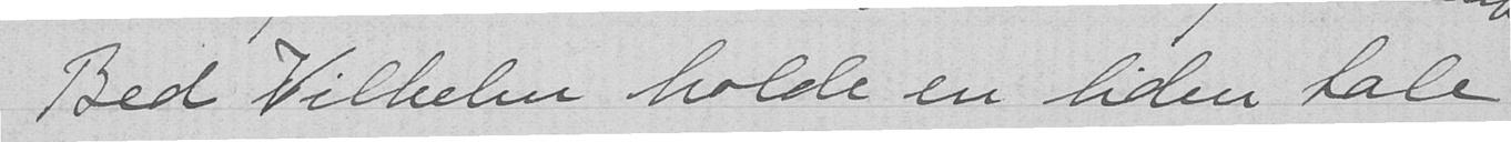Bed Vilhelm holde en liden tale
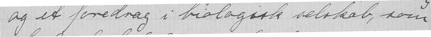og et foredrag i biologisk selskab, som
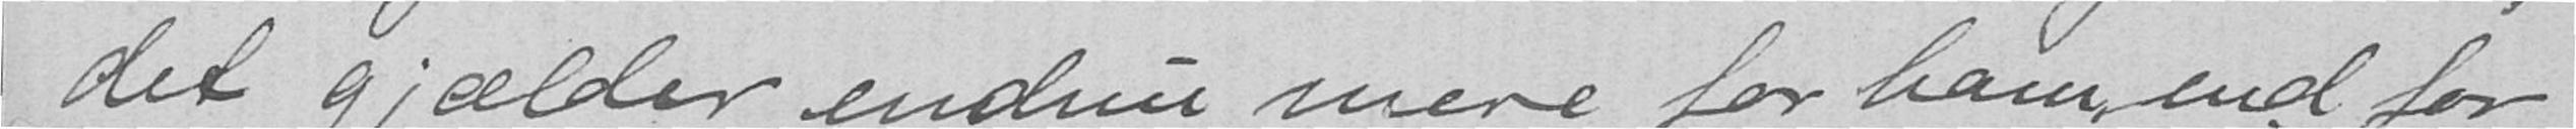det gjælder endnu mere for ham, end for
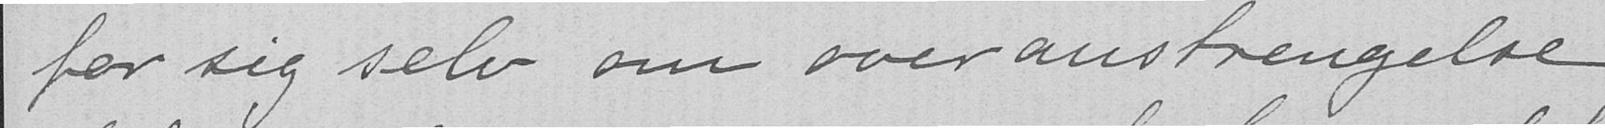for sig selv om overanstrængelse;
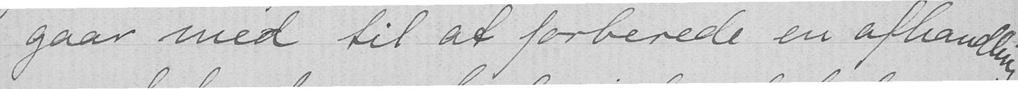gaar med til at forberede en afhandling
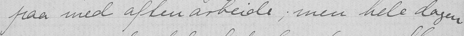paa med aftenarbeide; men hele dagen
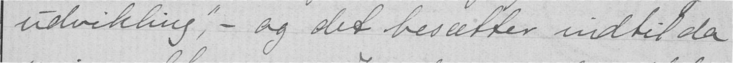udvikling" - og det besætter indtil da
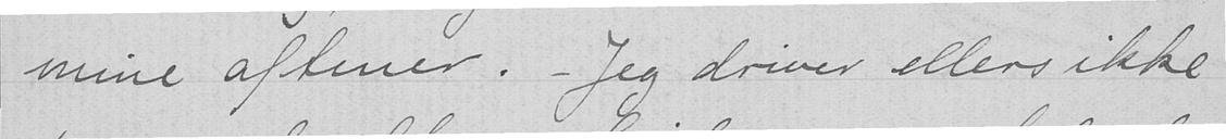mine aftener. - Jeg driver ellers ikke
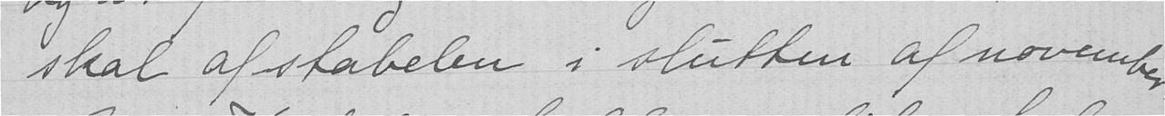skal af stabelen i slutten af november.
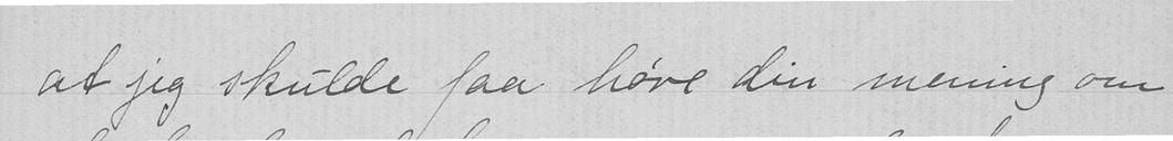at jeg skulde faa høre din mening om
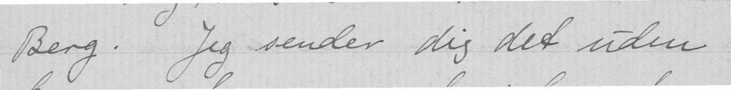Berg. Jeg sender dig det uden
